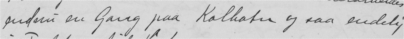endnu en Gang paa Kolbotn og saa endelig
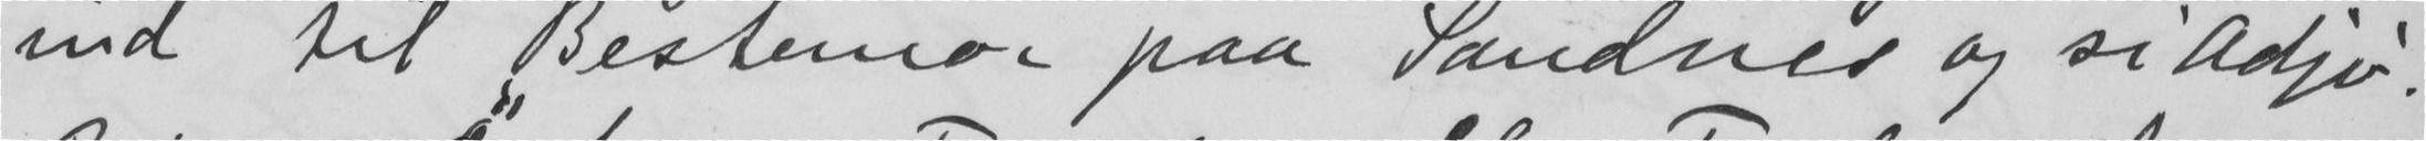ind til Bestemor paa Sandnes og si adjø.
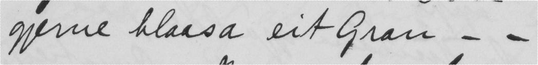gjerne blaasa eit Gran — —
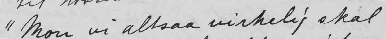"Mon vi altsaa virkelig skal
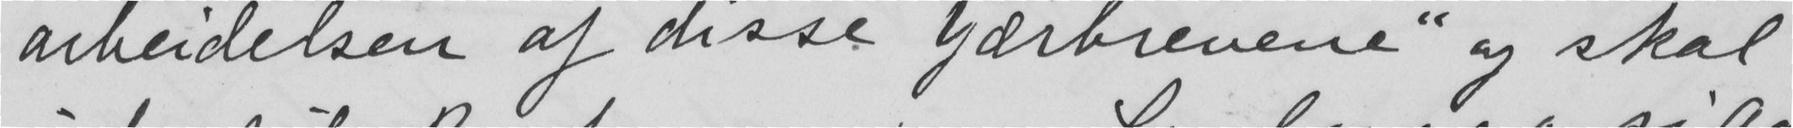arbeidelsen af disse Jærbrevene" og skal
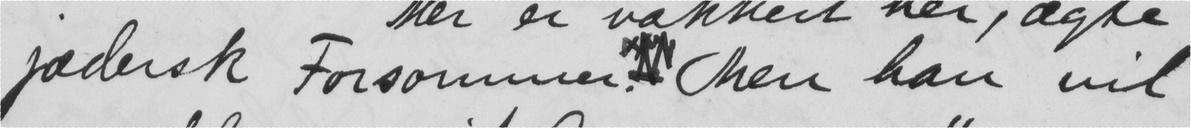jædersk Forsommer.  Men han vil
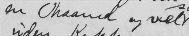en Maaned og vel
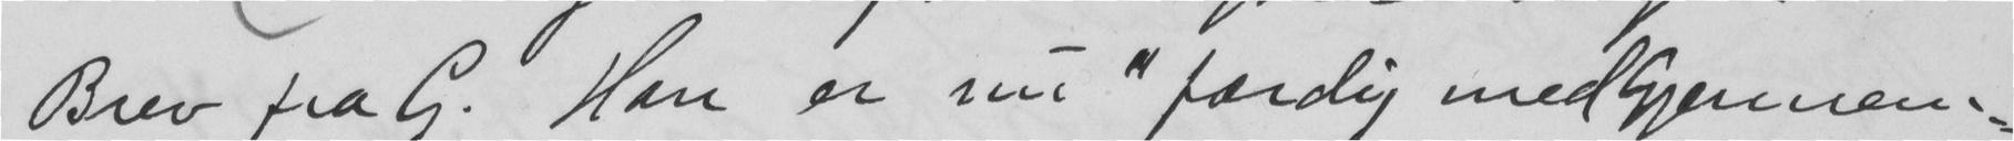Brev fra G. Han er nu "færdig med gjennem-
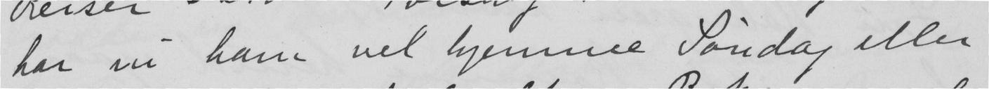har vi ham vel hjemme Søndag eller
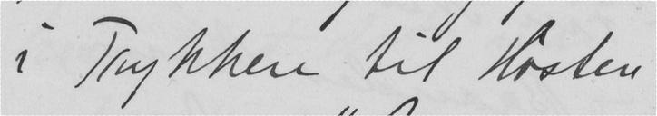i Trykken til Høsten
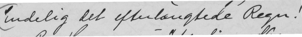Endelig det efterlængtede Regn!
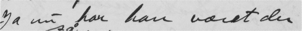Ja nu har han været der
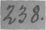238.
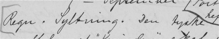Regn. Syltning. Den tyske
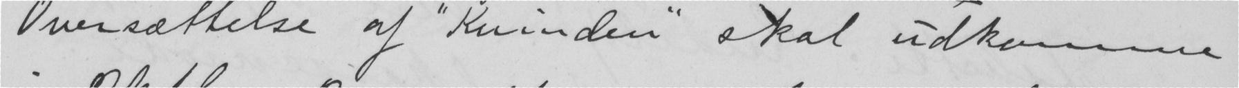Oversættelse af "Kvinden" skal udkomme
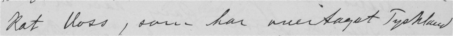kat Voss, som har overtagat Tyskland
